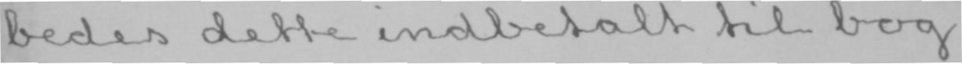bedes dette indbetalt til bog-
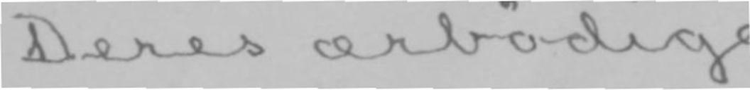Deres ærbødige
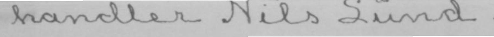handler Nils Lund.
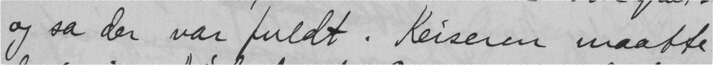og sa der var fuldt. Keiseren maatte
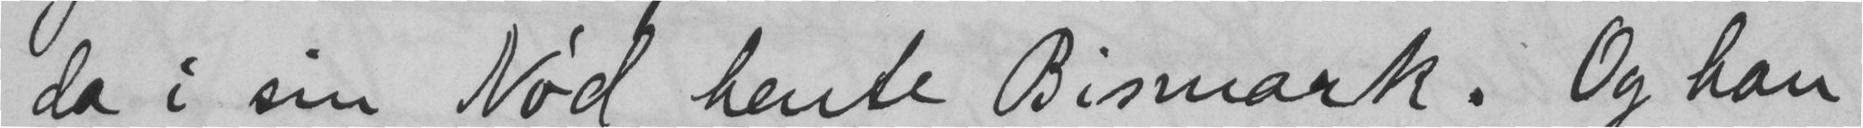da i sin Nød hente Bismark. Og han
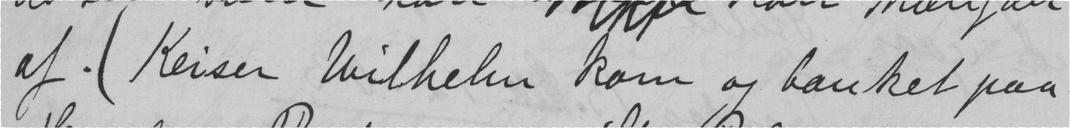af. (Keiser Wilhelm kom og banket paa
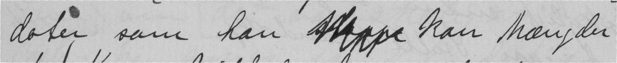døter som han  kan mængder
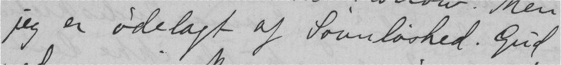jeg er ødelagt af Søvnløshed. Gud
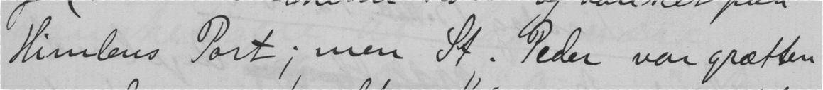Himlens Port; men St. Peder var grætten
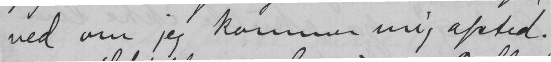ved om jeg kommer mig afsted.
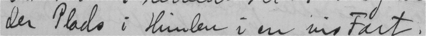der Plads i Himlen i en vis Fart.
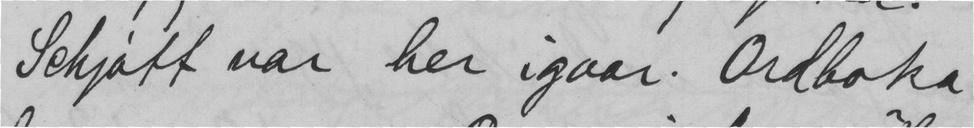Schjøtt var her igaar. Ordboka
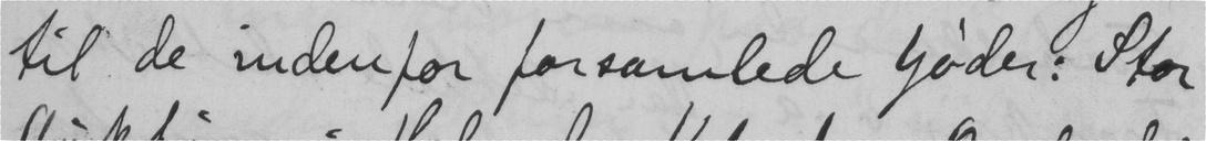til de indenfor forsamlede jøder: Stor
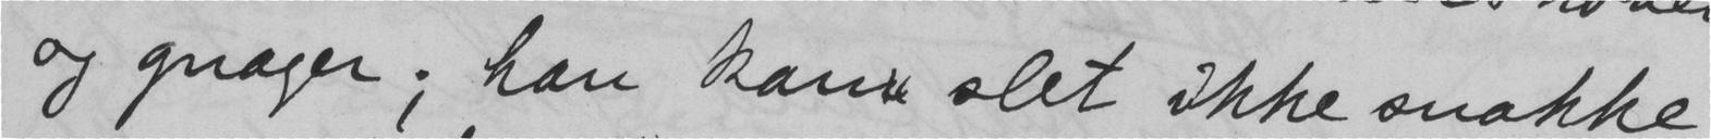og gnager; han kan slet ikke snakke
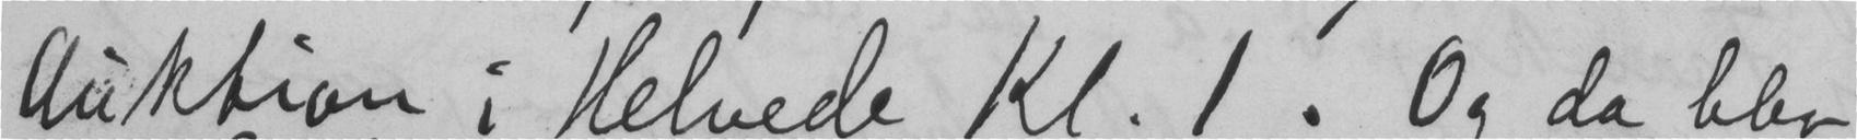auktion i Helvede Kl. 1. Og da blev
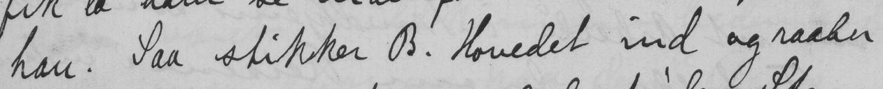han. Saa stikker B. Hovedet ind og raaber
